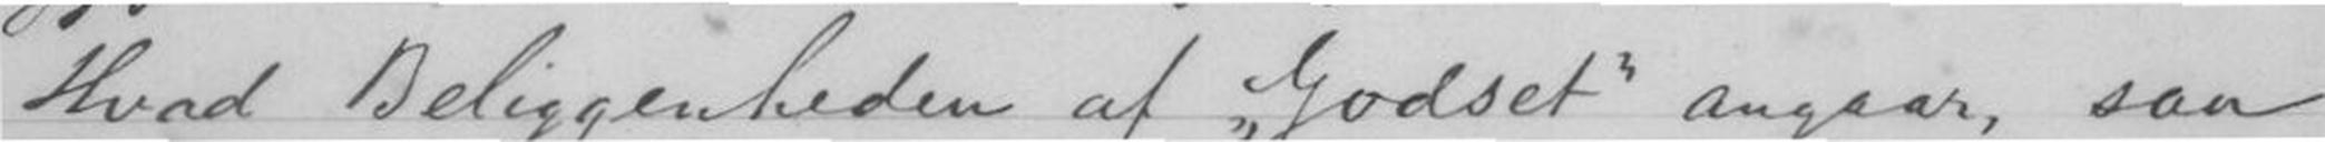Hvad Beliggenheden af Godset Angaar, saa
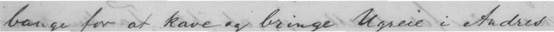bange for at kave og bringe Ugreie i Andres
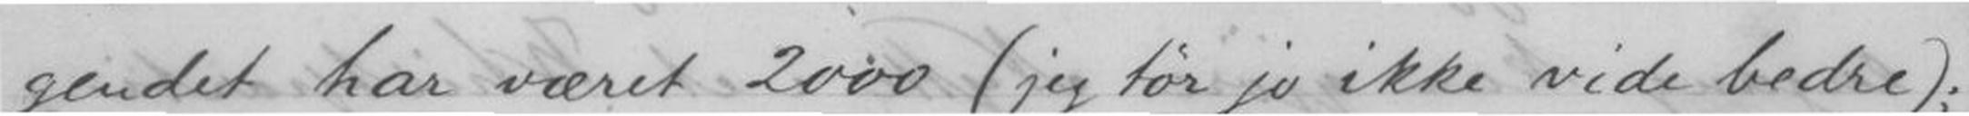gendet har været 2000 (jeg tør ikke vide bedre);
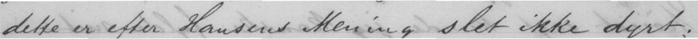dette er efter Hansens Mening slet ikke dyrt;
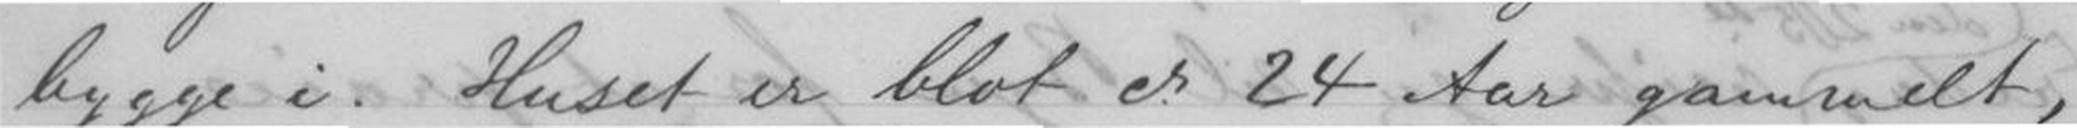bygge i. Huset er blot cr 24 Aar gammelt,
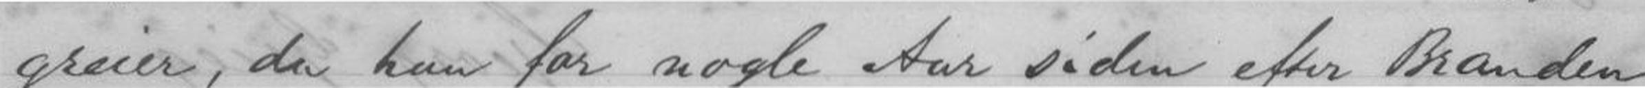greier, da kun for nogle Aar siden efter Branden
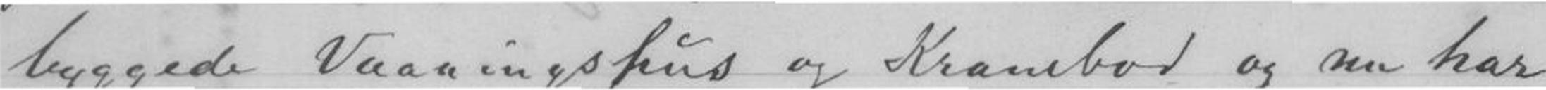byggede Vaaningshus og Krambod og nu har
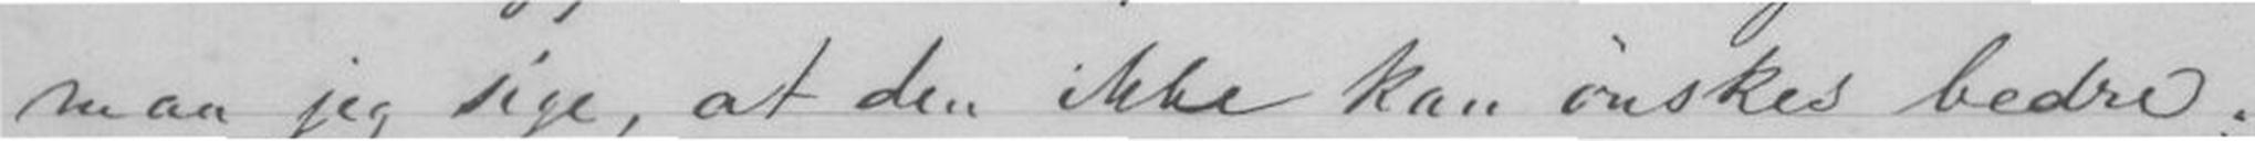maa jeg sige, at den ikke kan ønske bedre;
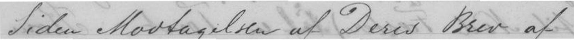Siden modtagelsen af Deres Brev af
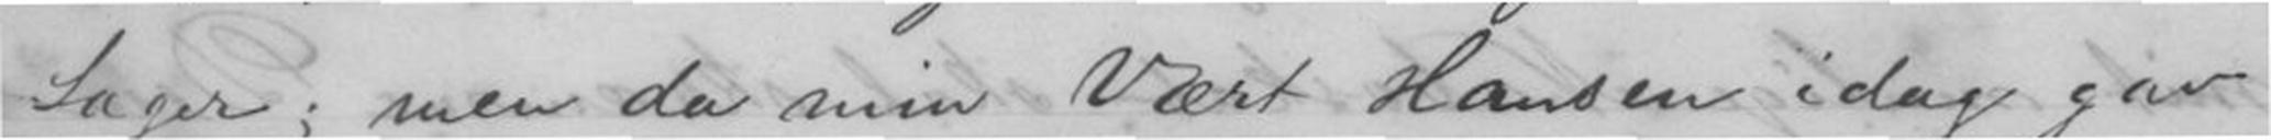Sager; men da min Vært Hansen idag gav
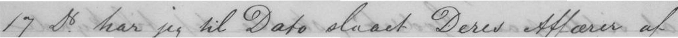17D. har jeg til Dato slaaet Deres Affærer af
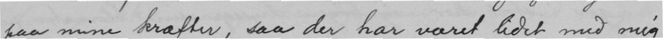paa mine kræfter, saa der har været lidet med mig
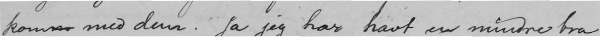kom med dem. Ja jeg har havt en mindre bra
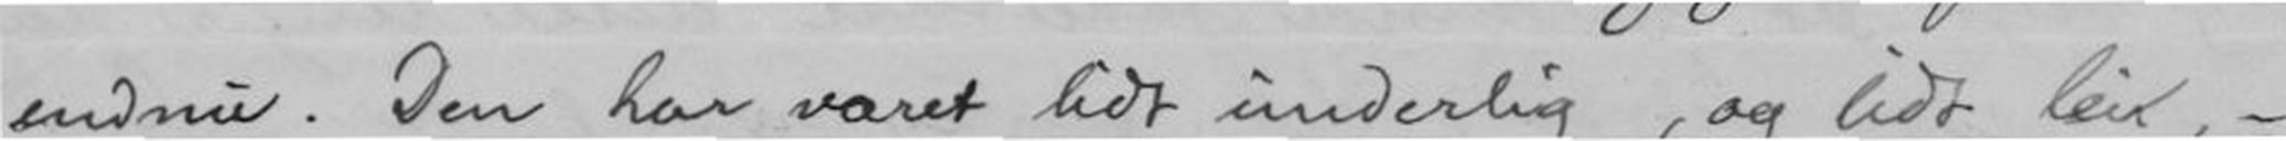endnu. Den har været lidt underlig, og lidt leit,-
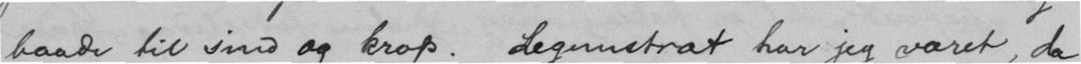baade til sind og krop. Legemstræt har jeg været, da
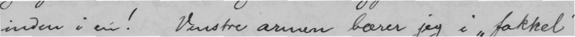inden i en! Venstre ermen bærer jeg i "fakkel"
